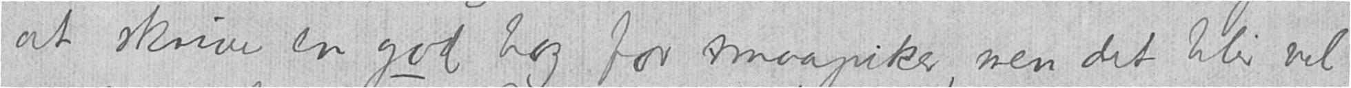at skrive en god bog for smaapiker, men det blir vel
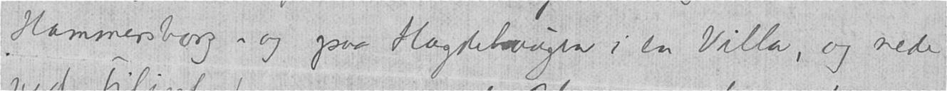Hammersborg - og paa Hægdehaugen i en Villa, og nede
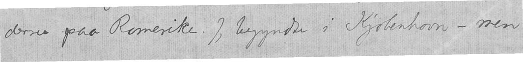derne paa Romerike. Jeg begyndte i Kjøbenhavn – men
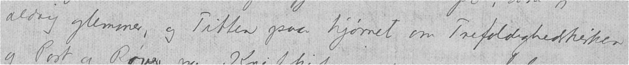aldrig glemmer, og Titten paa hjørnet om Trefoldighedskirken
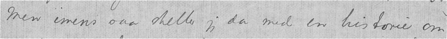Men imens saa steller jeg da med en historie om
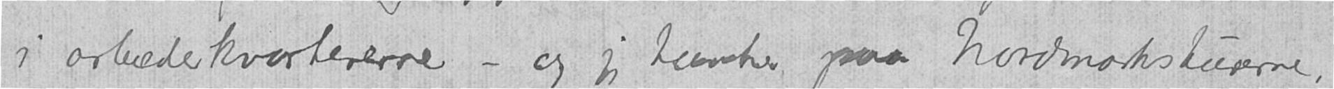i arbeiderkvartererne - og jeg tænker paa Nordmarksturerne,
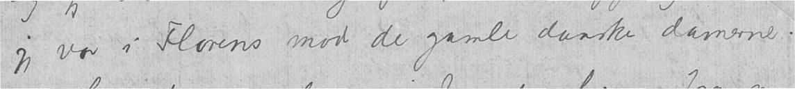jeg var i Florens mod de gamle danske damerne.
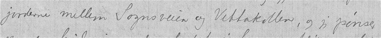jorderne mellem Sognsveien og Vettakollen, og jeg pønser
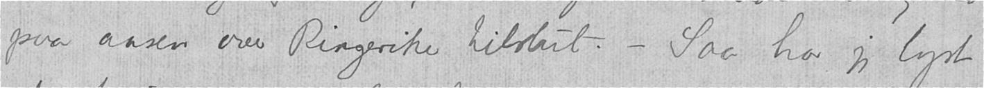paa aasen over Ringerike tilslut. - Saa har jeg lyst
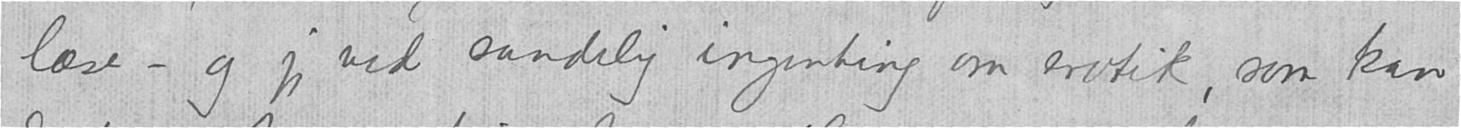læse - og jeg ved sandelig ingenting om erotik, som kan
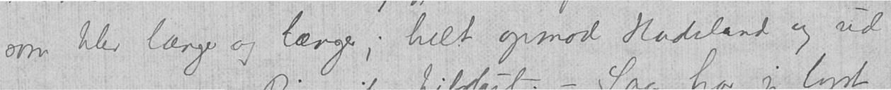som blev længer og længer; helt opmod Hadeland og ud
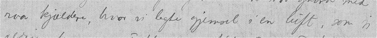raa kjældere, hvor vi legte gjemsel i en luft, som jeg
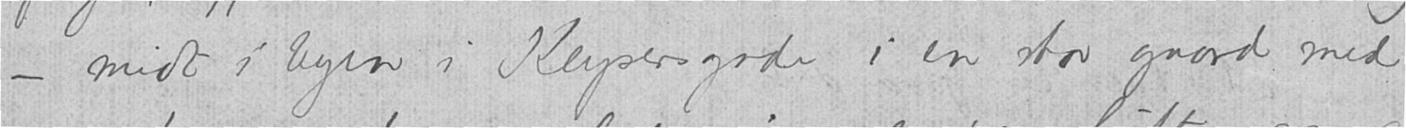- midt i byen i Keysersgade i en stor gaard med
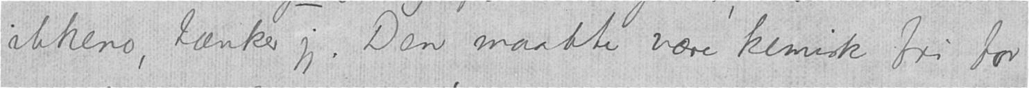ikkeno, tænker jeg. Den maatte være kemisk fri for
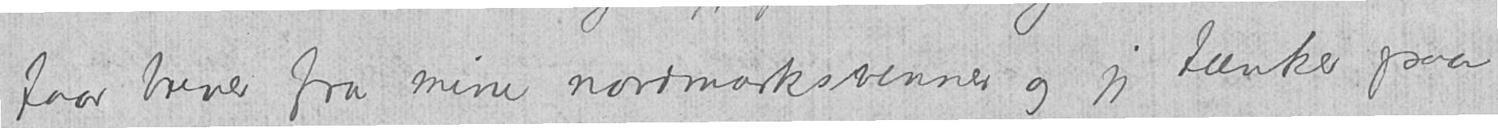faar brever fra mine nordmarksvenner og jeg tænker paa
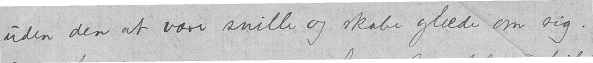uden den at være snille og skabe glæde om sig.
